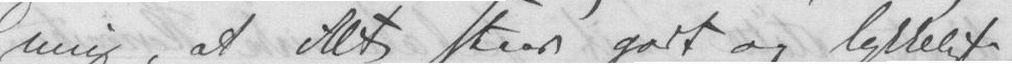mig, at Alt godt og lykkeligt
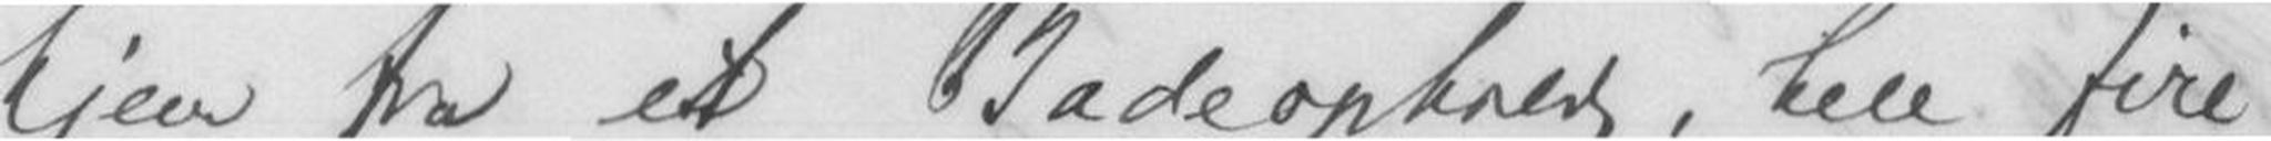hjem fra et Badeophold, hele fire
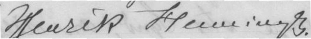Henrik Hennings.
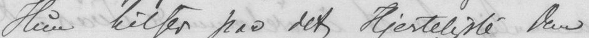Hun hilser paa det Hjerteligste Dem
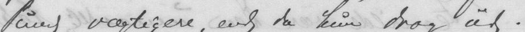vægtigere, end da hun drog ud.
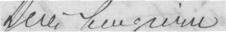Deres hengivne
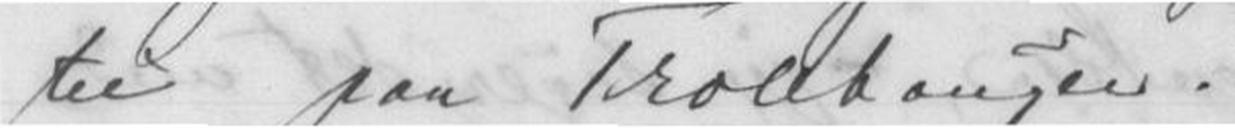til paa Troldhaugen.
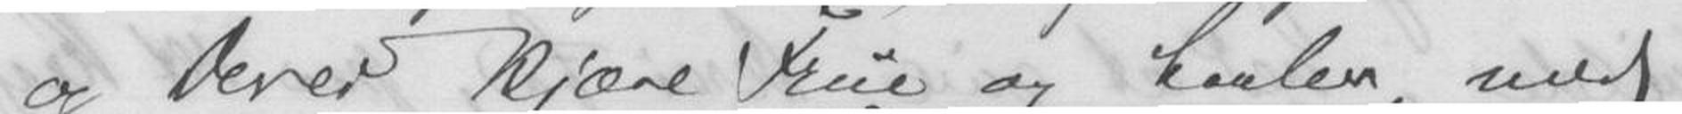og Deres kjære Frue og haaber, med
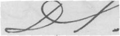D.S.
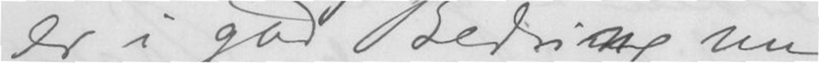er i god Bedring nu
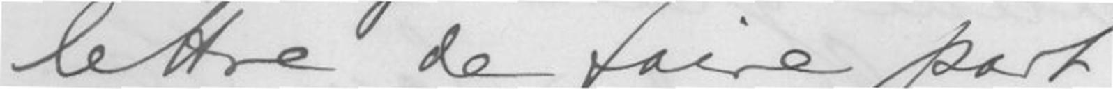lettre de faire part.
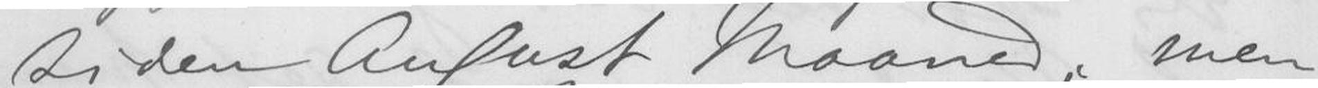siden August Maaned; men
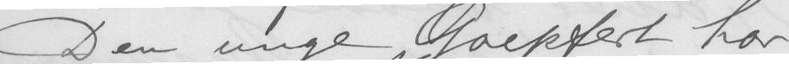Den unge Gaepfert har
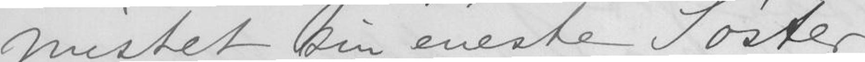mistet sin eneste Søster,
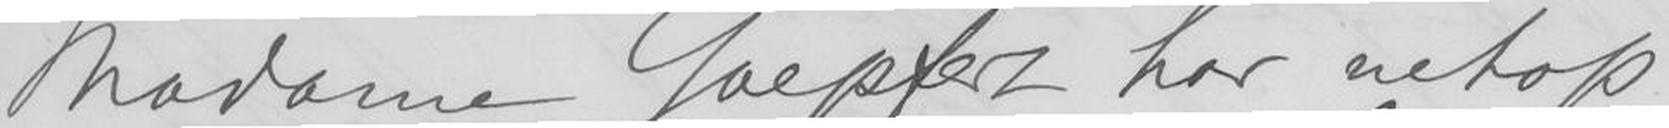Madame Gaepfert har netop
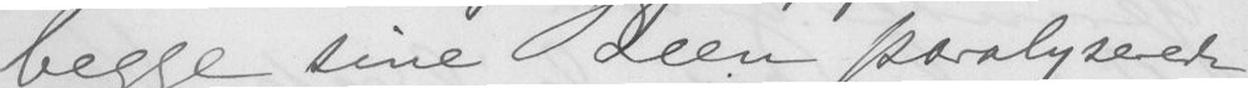begge sine Been paralyserede
